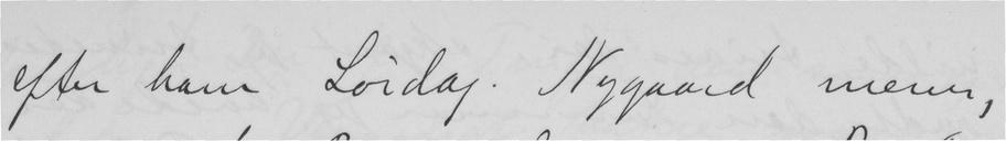efter ham Lørdag. Nygaard mener,
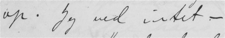op? Jeg ved intet —
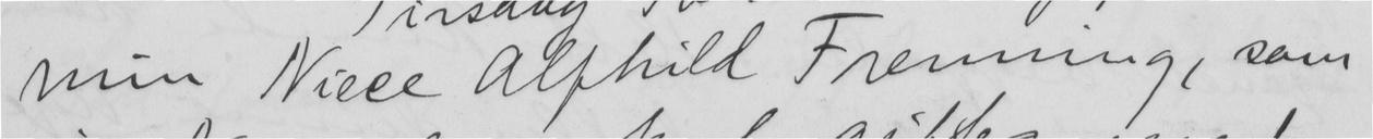min Niece Alfhild Frenning, som
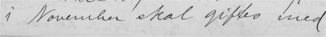i November skal giftes med
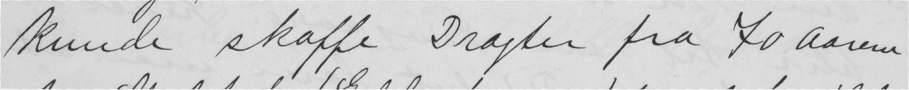kunde skaffe Dragten fra 70 Aarene
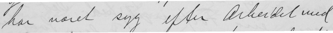har været syg efter Arbeidet med
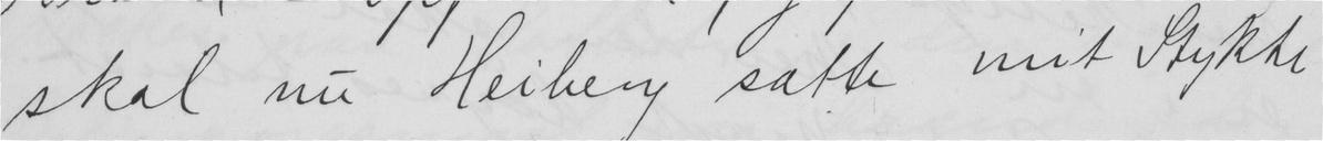skal nu Heiberg sætte mit Stykke
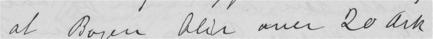at Bogen blir over 20 Ark
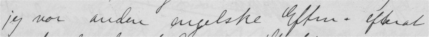Jeg vor anden engelske Eften. efterat
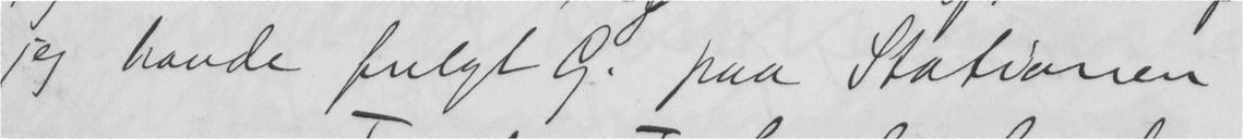jeg havde fulgt G. paa Stationen
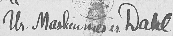Hr. Maskinmester Dahl
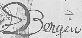Bergen
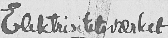Elektrisitetsværket
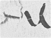til
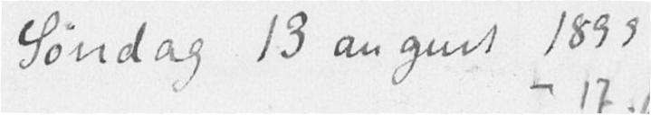Søndag 13 august 1899
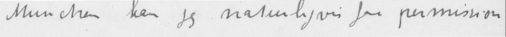Munchen kan jeg naturligvis faa permission
